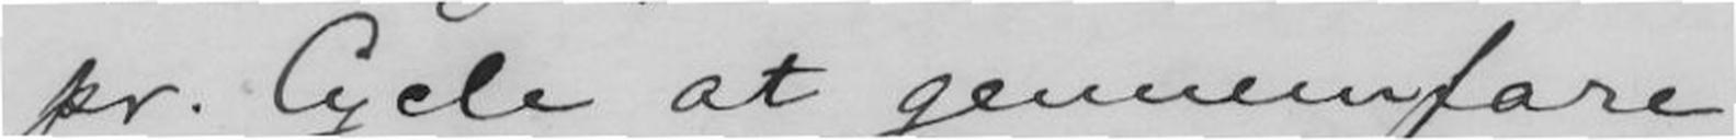pr. Cycle at gennemfare
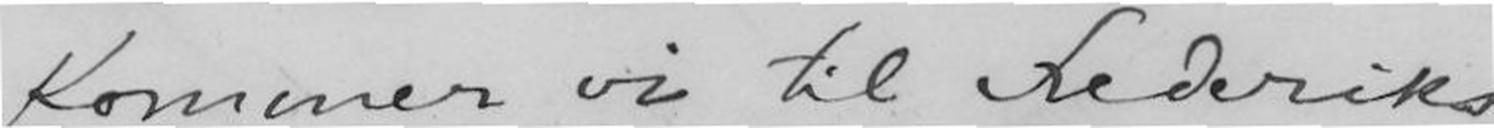kommer vil til Frederiks-
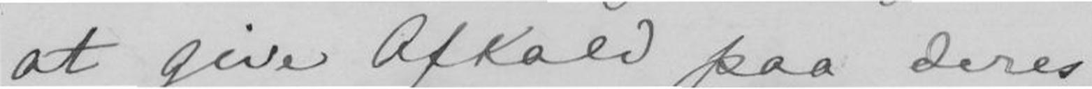at give Afkald paa Deres
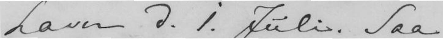havn d. 1. Juli. Saa
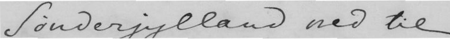Sønderjylland ned til
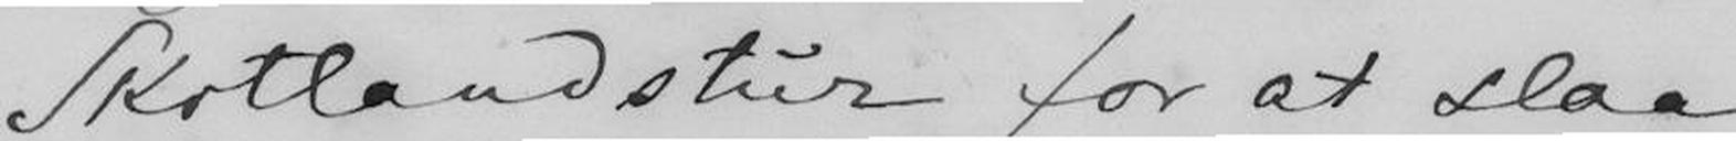Skotlandstur for at slaa
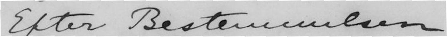Efter Bestemmelsen
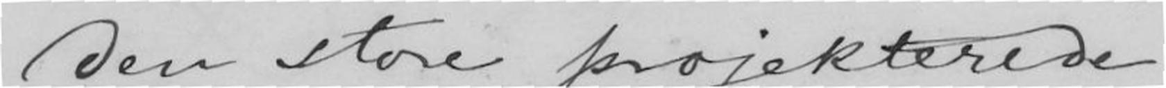Den store projekterede
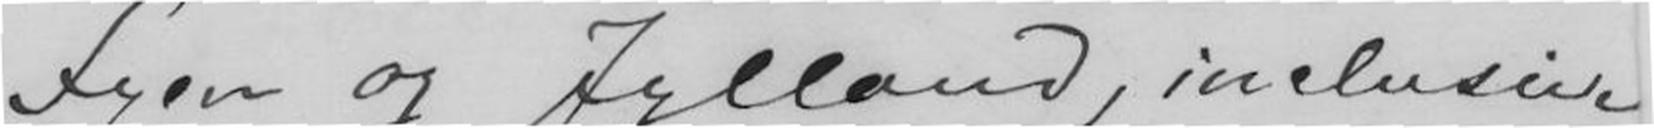Fyen og Jylland, inclusive
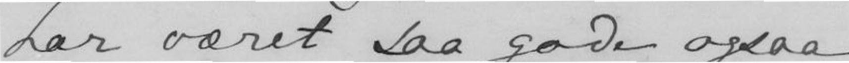har været saa gode ogsaa
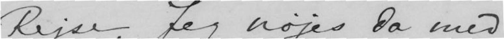Rejse. Jeg nøjes da med
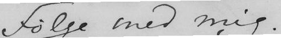følge med mig.
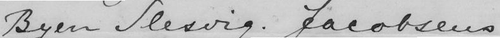Byen Slesvig. Jacobsens
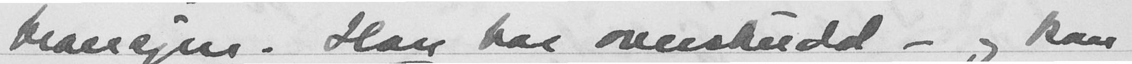Mangen. Han har overskudd - og kan
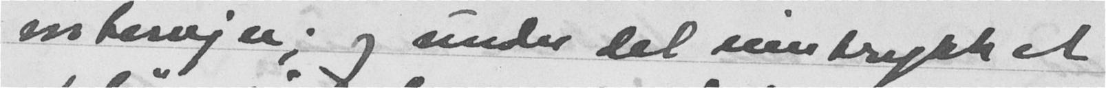intervju; og under det inntrykket
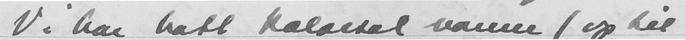Vi har hatt kolossal varme (op til
